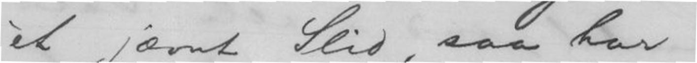et jævnt Slid, saa har
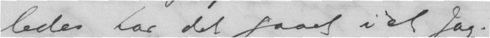ledes har det gaaet i et Jag.
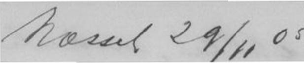Næsset 29/11 05
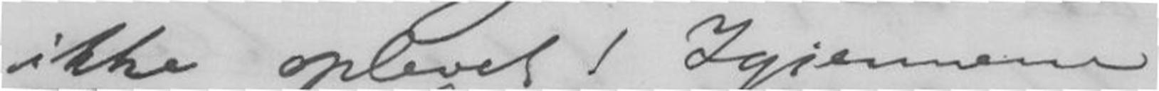ikke oplevet! Igjennem
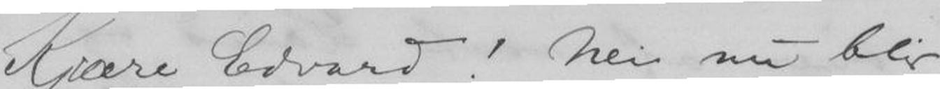Kjære Edvard! Nei nu blir
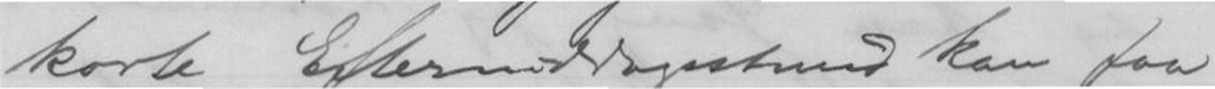korte Eftermiddagsstund kan faa
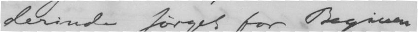derinde sørget for Begiven-
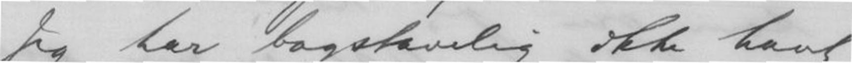Jeg har bogstavelig ikke havt
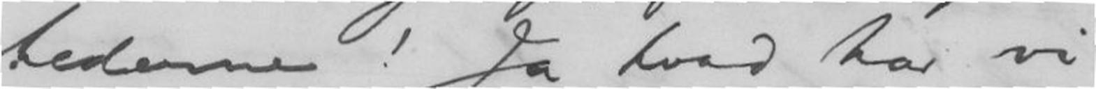hederne! Ja hvad har vi
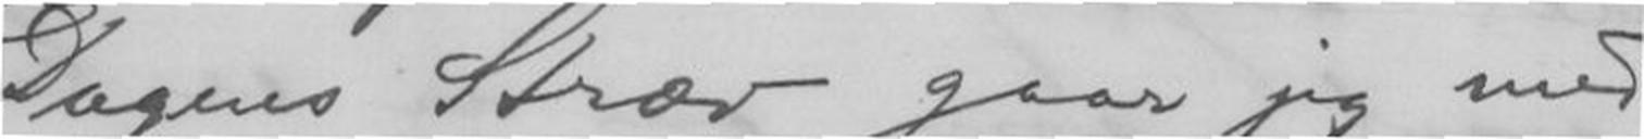Dagens Stræv gaar jeg med
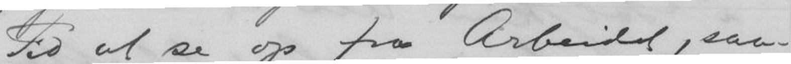Tid at se op fra Arbeide, saa-
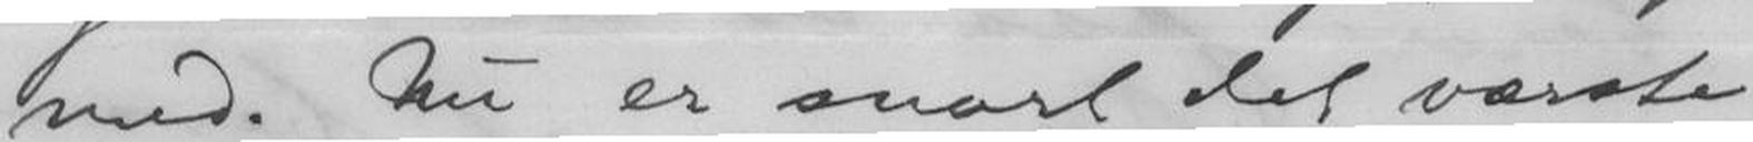med. Nu er snart det værste
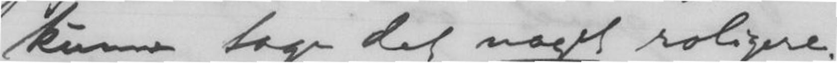kunne tage det noget roligere.
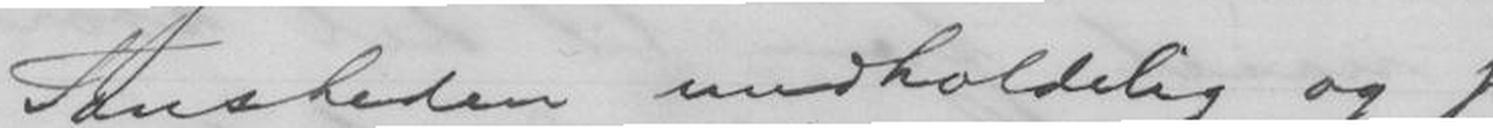Tausheden uudholdelig og jeg
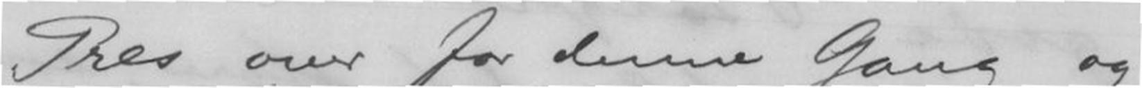Pres over for denne gang og
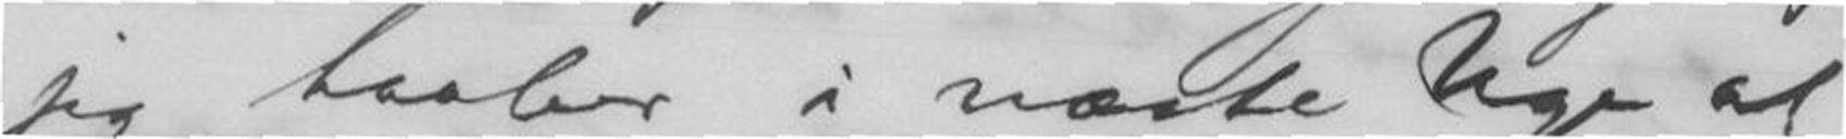jeg haaber i næste Uge at
